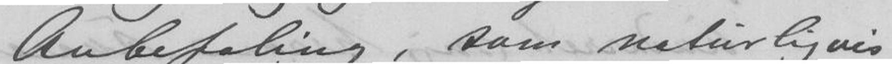Anbefaling, som naturligvis
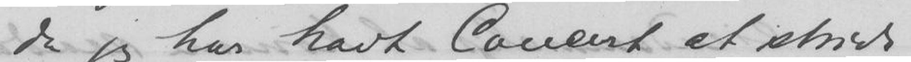da jeg har havt Concert at stride
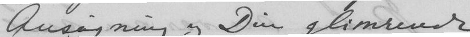Ansøgning og Din glimrende
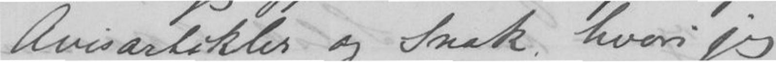Avisartikler og Snak hvori jeg
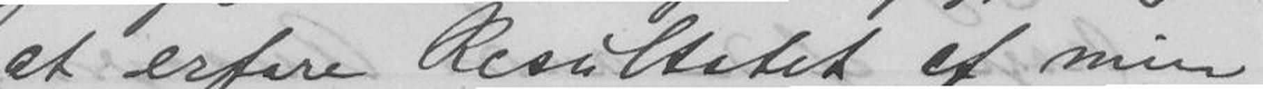at erfare Resultatet af min
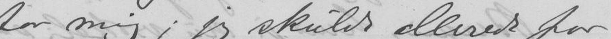for mig; jeg skulde allerede for
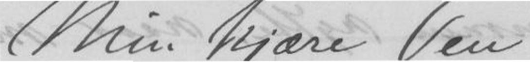Min kjære Ven!
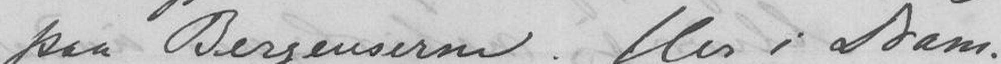paa Bergenserne. Her i Dram-
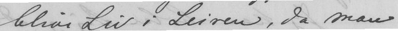blive Liv i Leiren, da man
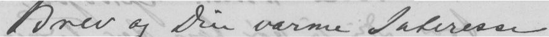Brev og Din varme Interesse
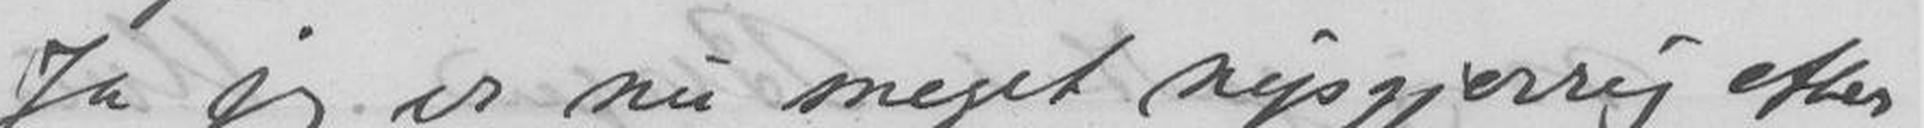Ja jeg er nu meget nysgjerrig efter
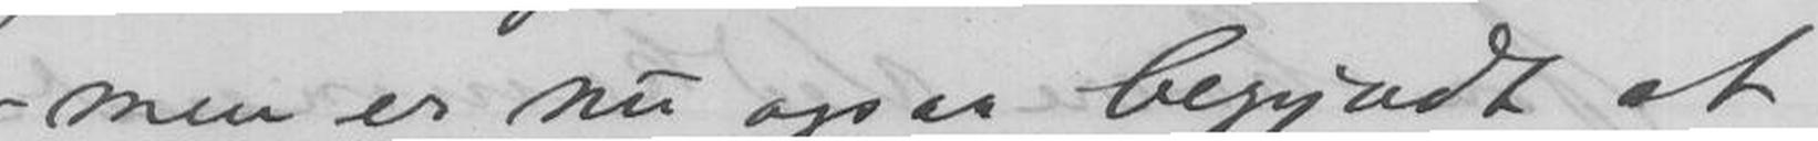men er nu ogsaa begyndt at
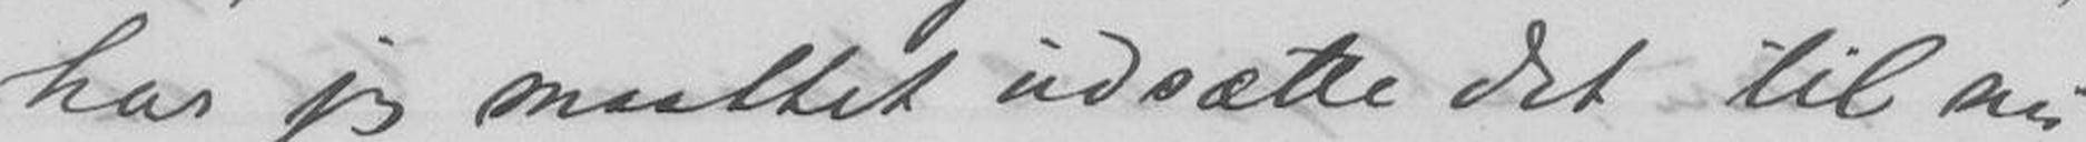har jeg maattet udsætte det til nu.
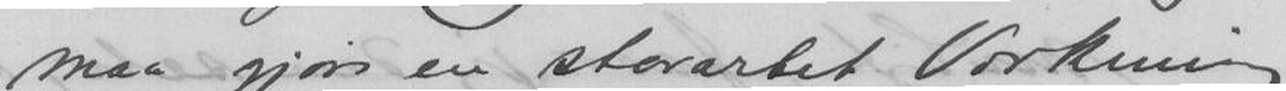maa gjøre en storartet Virkning
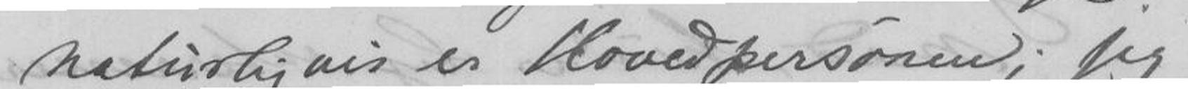naturligvis er Hovedpersónen; jeg
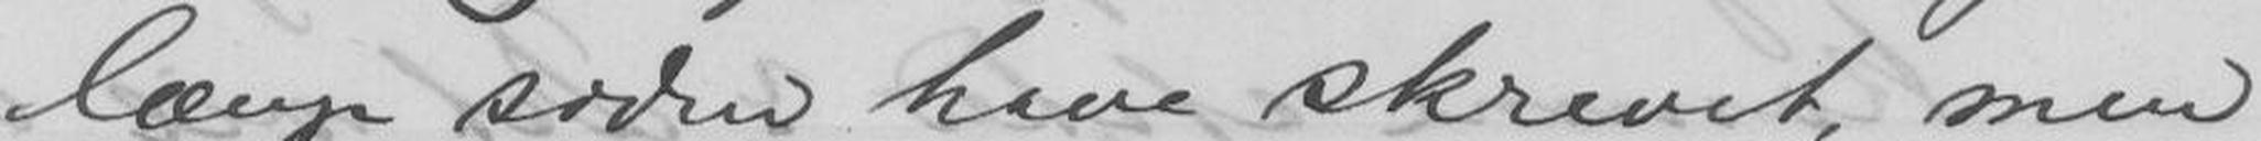længe siden have skrevet, men
